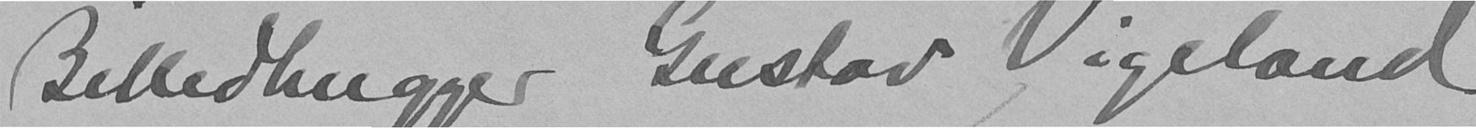Billedhugger Gustav Vigeland
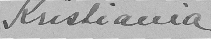Kristiania
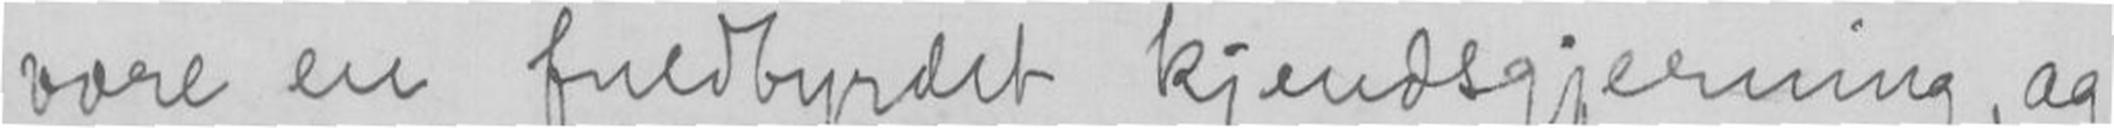være en fuldbyrdet kjendsgjerning, og
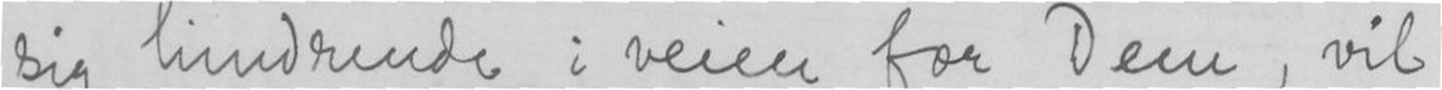sig hindrende i veien for Dem, vil
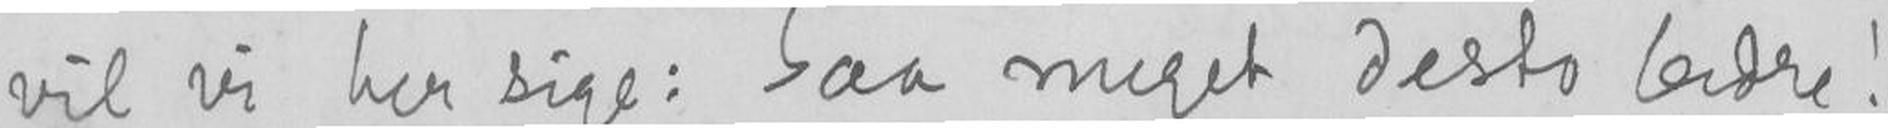vil vi her sige: Saa meget desto bedre!
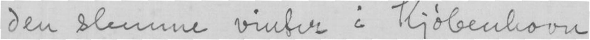den slemme vinter i Kjøbenhavn
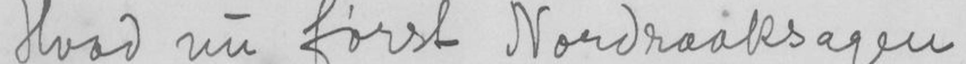Hvad nu først Nordraaksagen
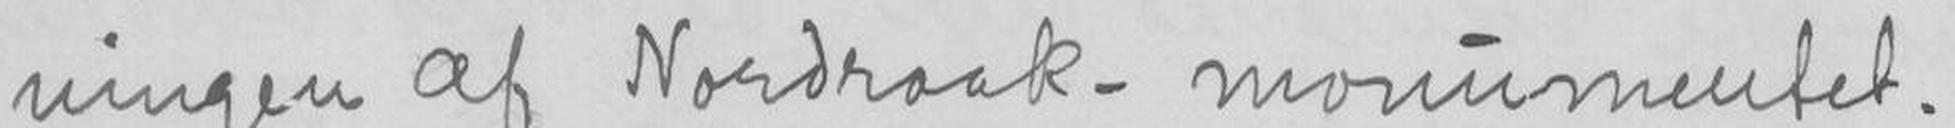ningen af Nordraak-monumentet.
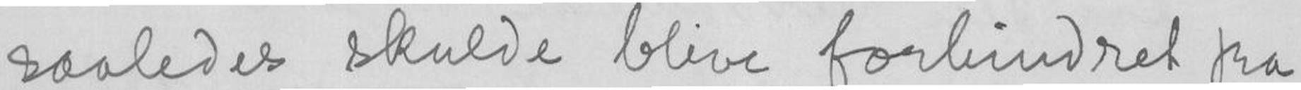saaledes skulde blive forhindret fra
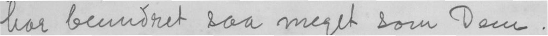har beundret saa meget som Dem.
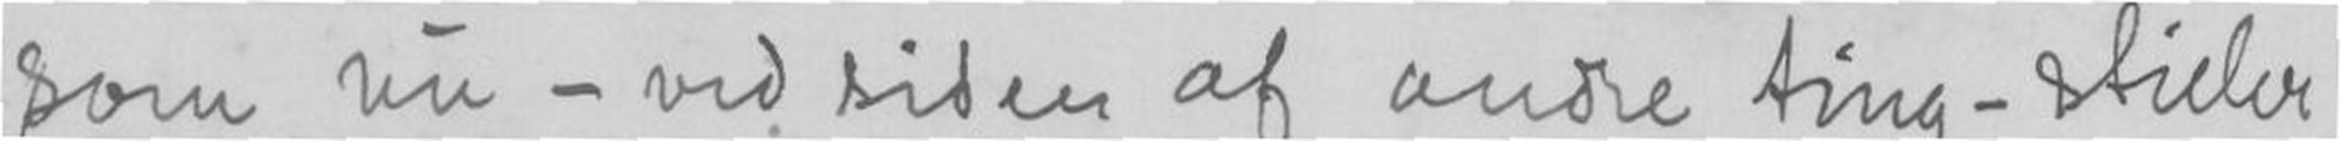som nu - ved siden af andre ting - stiller
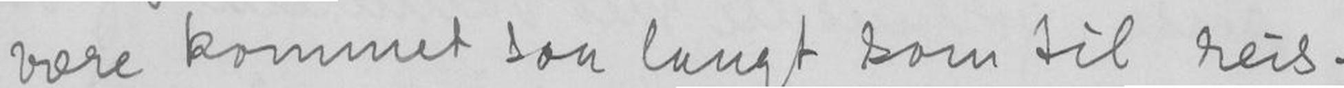være kommet saa langt som til reis-
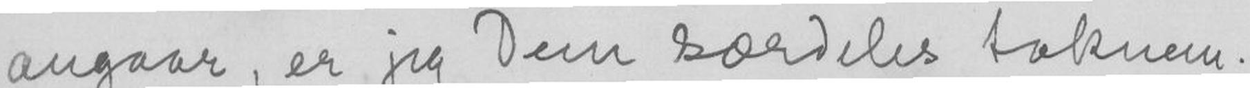angaar, er jeg Dem særdeles taknem-
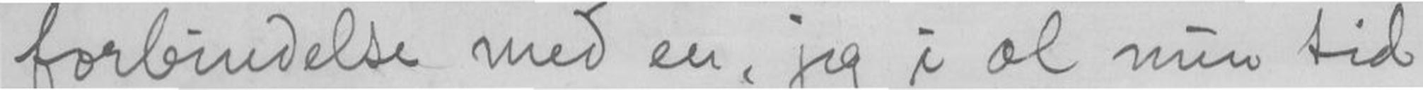forbindelse med en, jeg i al min tid
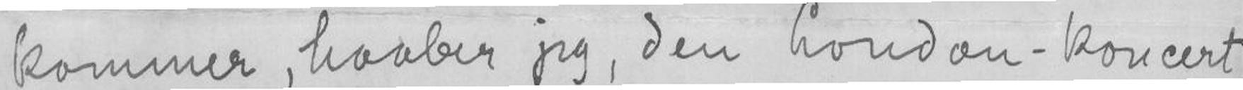kommer, haaber jeg, den London-concert,
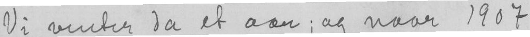Vi venter da et aar; og naar 1907
